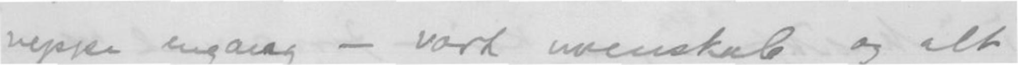neppe engang - vort uvenskab og alt
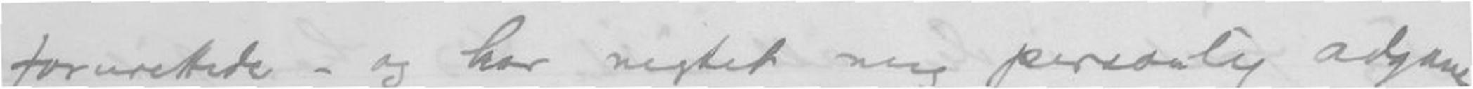forurettede - og har negtet mig personlig adgang
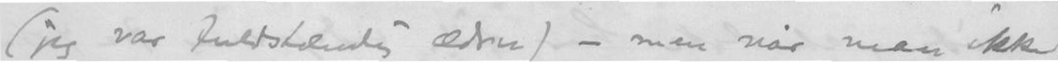(jeg var fuldstændig ædru) - men når man ikke
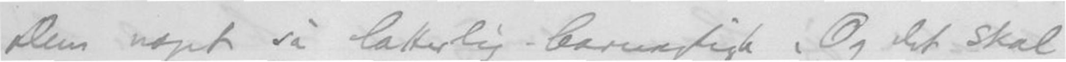Dem noget så latterlig barnsligt. Og det skal
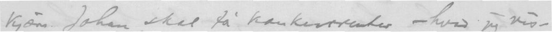kjære Johan skal få konkurrenter - hvad jeg vis-
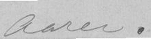Aarer.
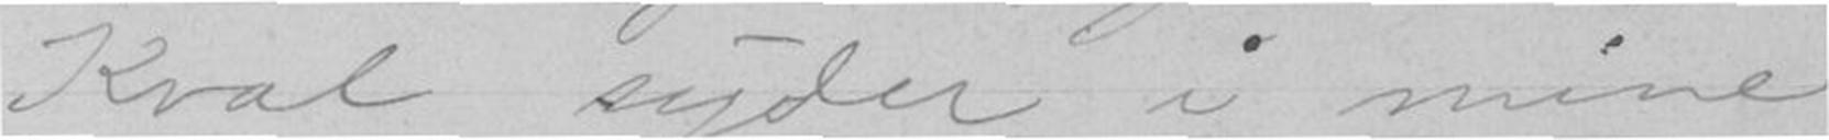Kval syder i Mine
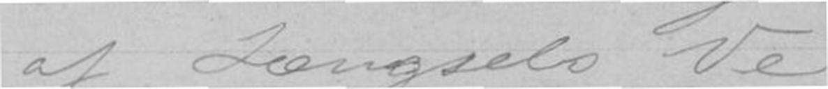af længsels Ve.
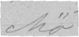Mø
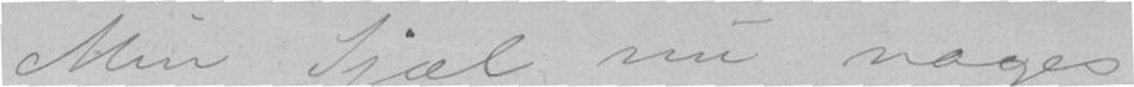Min Sjæl nu nages
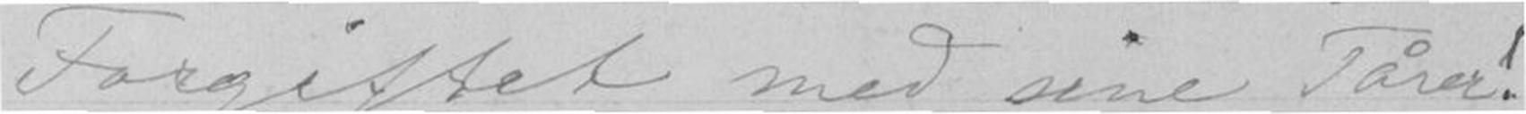Forgiftet med sine Tårer!
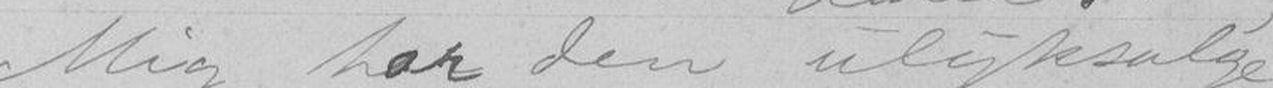Mig har den ulyksal'ge
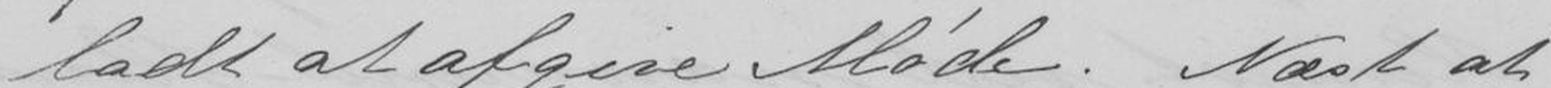ladt at afgive Møde. Næst at
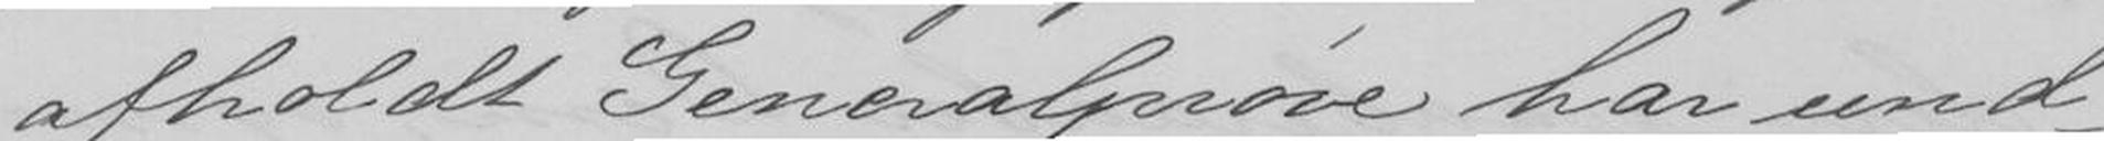afholdt Generalprøve har und-
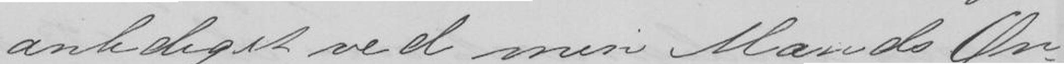anlediget ved min Mands Øn-
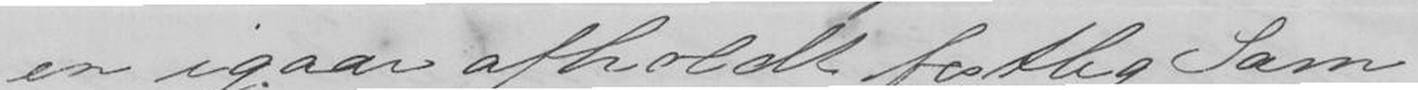en igaar afholdt festlig Sam-
